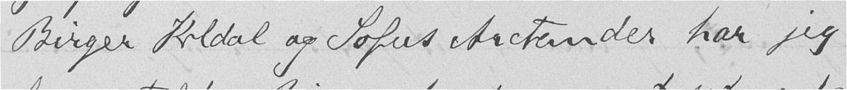Birger Kildal og Sofus Arctander har jeg
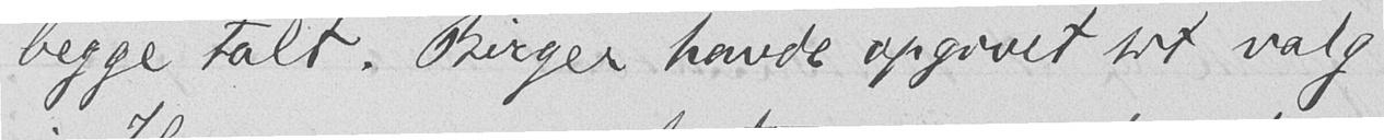begge talt. Birger havde opgivet sit valg
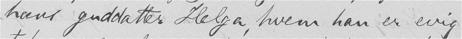hans guddatter Helga, hvem han er evig
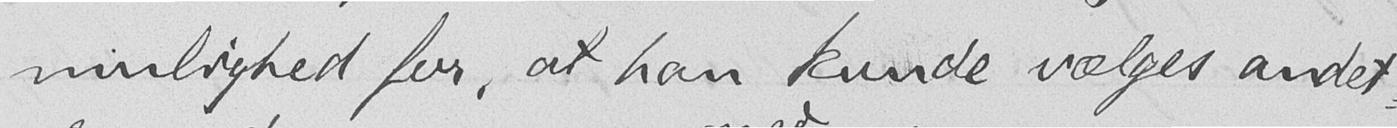mulighed for, at han kunde vælges andet-
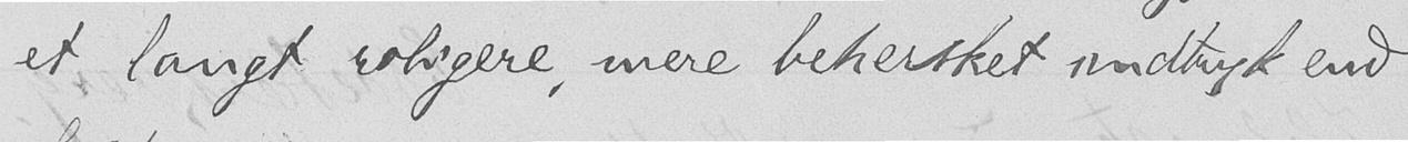et langt roligere, mere behersket indtryk end
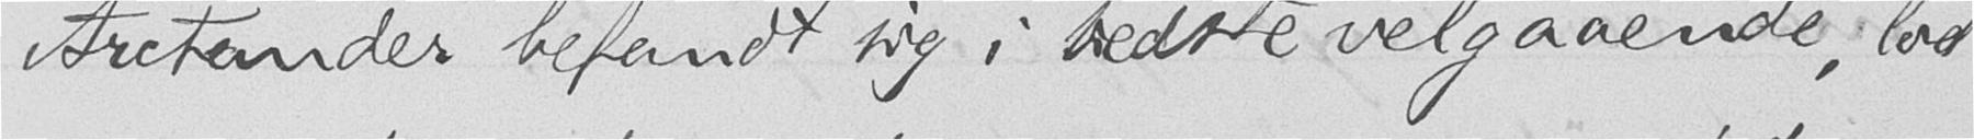Arctander befandt sig i bedste velgaaende, lod
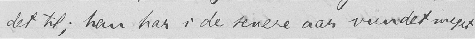det til; han har i de senere aar vundet meget
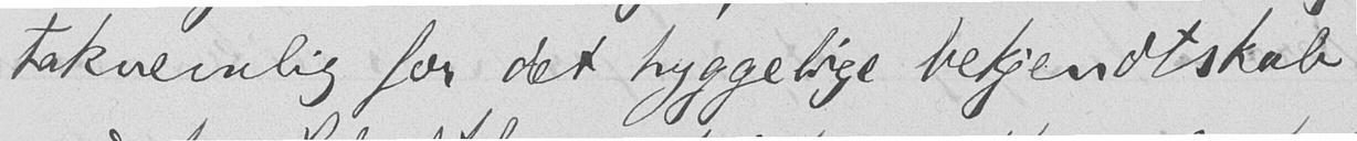taknemlig for det hyggelige bekjendtskab
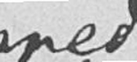med
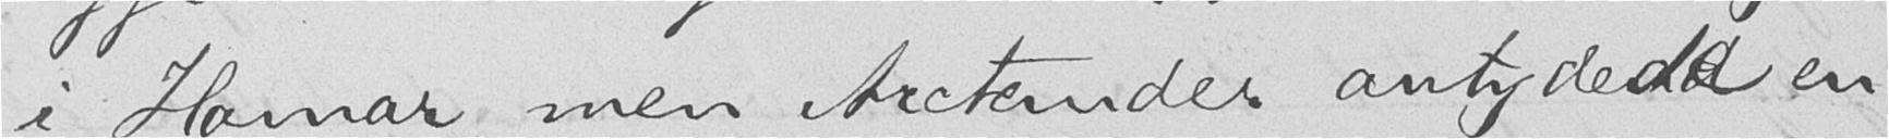i Hamar, men Arctander antydede en
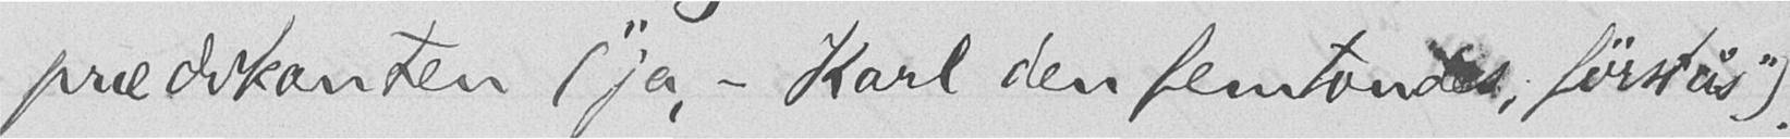predikanten ("ja, – Karl den femtondes, førstås").
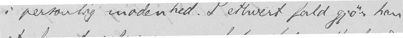i personlig modenhed. I ethvert fald gjør han
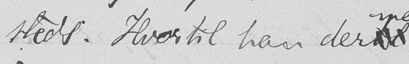steds. Hvortil han dertil
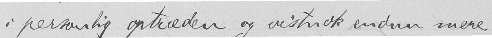i personlig optræden og vistnok endnu mere
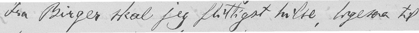Fra Birger skal jeg flittigst hilse, ligesaa til
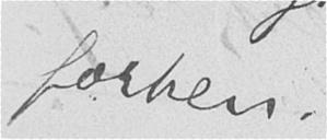forhen.
